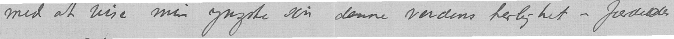med at vise min yngste søn denne verdens herlighet – færdedes
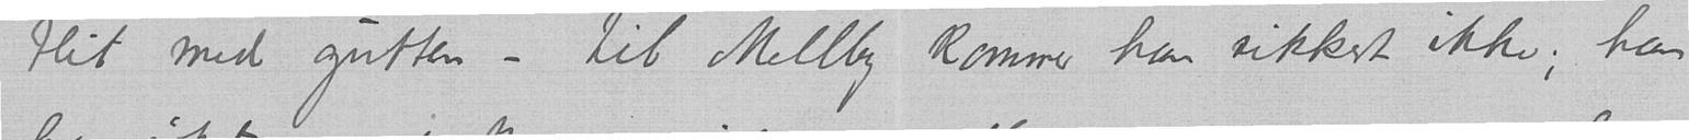blit med gutten – til Mellby kommer han sikkert ikke; han
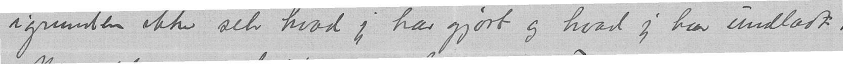igrunden ikke selv hvad jeg har gjort og hvad jeg har undladt.
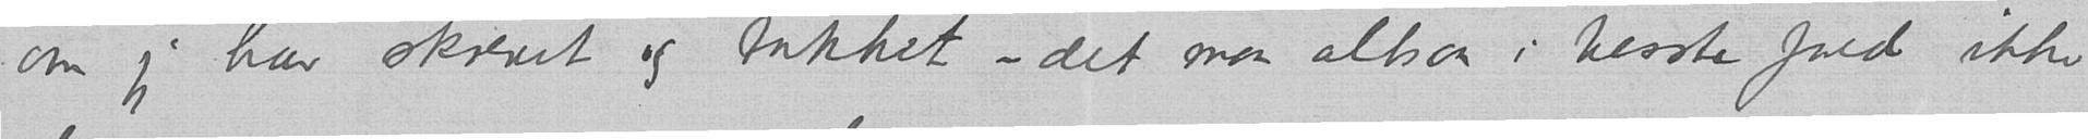om jeg har skrevet og takket – det maa altsaa i besste fald ikke
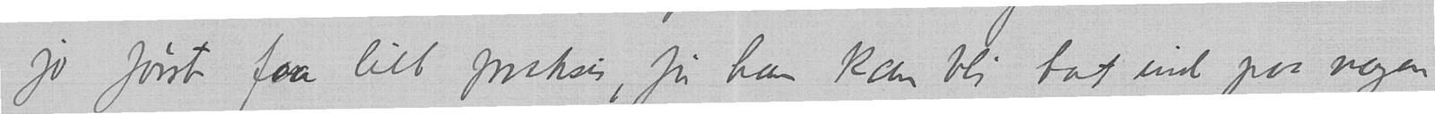jo først faa litt praksis, før han kan bli tat ind paa nogen
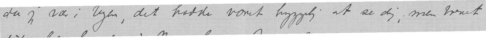da jeg var i byen, det hadde været hyggelig at se dig, men brevet
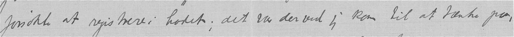forsøkte at registrere i hodet; det var derved jeg kom til at tænke paa,
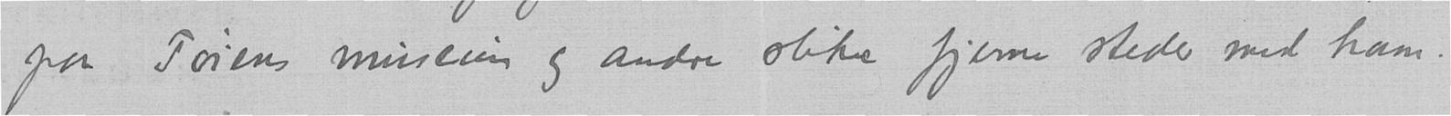paa Tøiens museum og andre slike fjerne steder med ham.
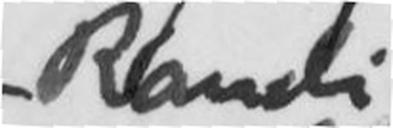Randi
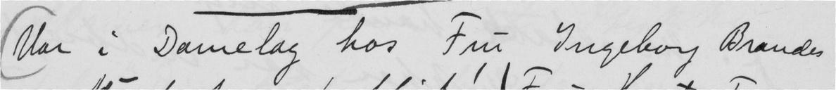Var i Damelag hos Fru Ingeborg Brandes
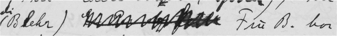Blehr)  Fru B. bor
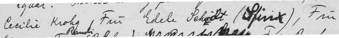Cecilie Krohg, Fru Edele Schødt (Sfinx), Fru
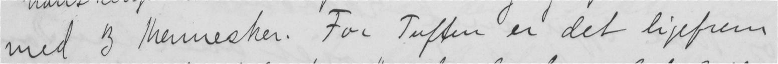med 3 Mennesker. For Tuften er det ligefrem
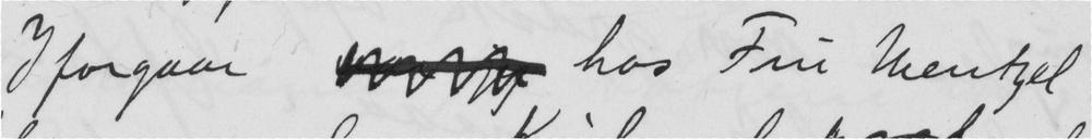Iforgaar  hos Fru Wentzel
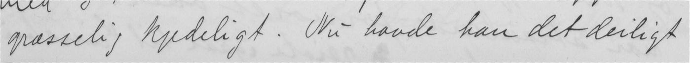græsselig kjedeligt. Nu havde han det deiligt
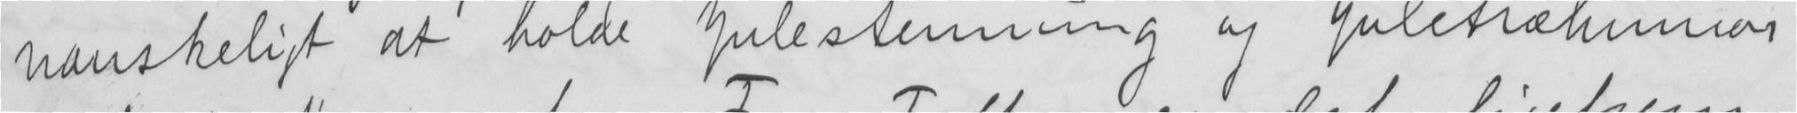vanskeligt at holde Julestemning og Juletræhumør
